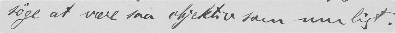søge at være saa objektiv som muligt.
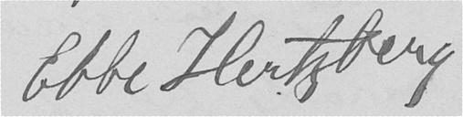Ebbe Hertzberg
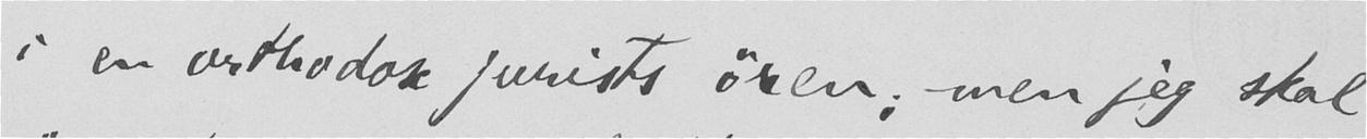i en orthodox jurists øren; men jeg skal
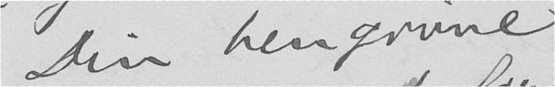Din hengivne
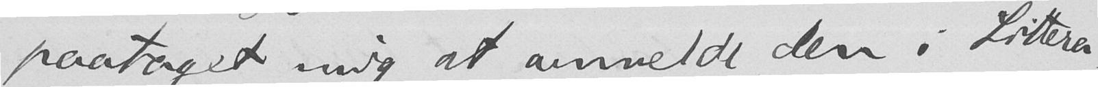paataget mig at anmelde den i Littera-
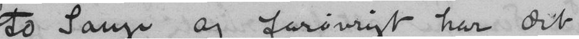to Sange og forøvrigt har det
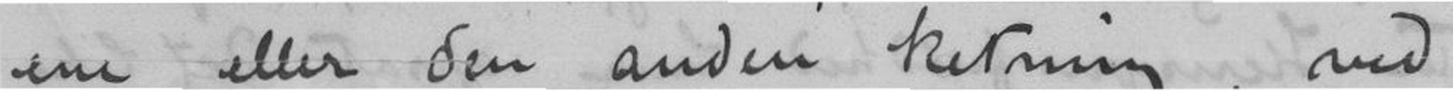ene eller den anden Retning, ved
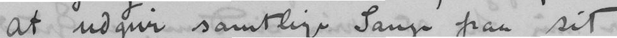at udgive samtlige Sange paa sit
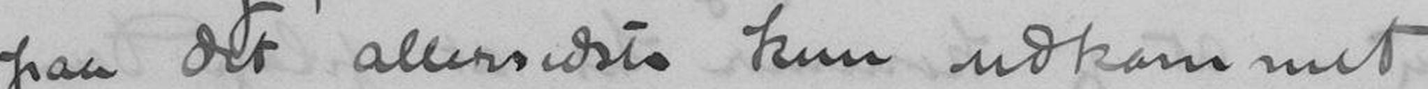paa det allersidste kun udkommet
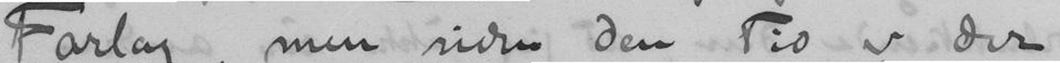Forlag, men siden den Tid er der
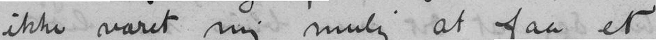ikke varet mig mulig at faa et
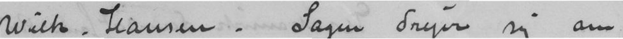Wilh. Hansen. Sagen drejer sig om
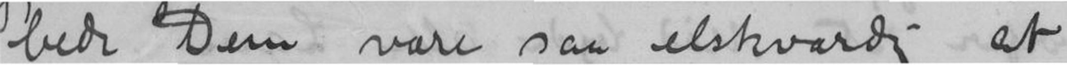bede Dem vare saa elskværdig at
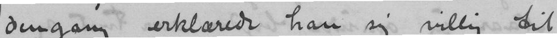dengang erklærede han sig villig til
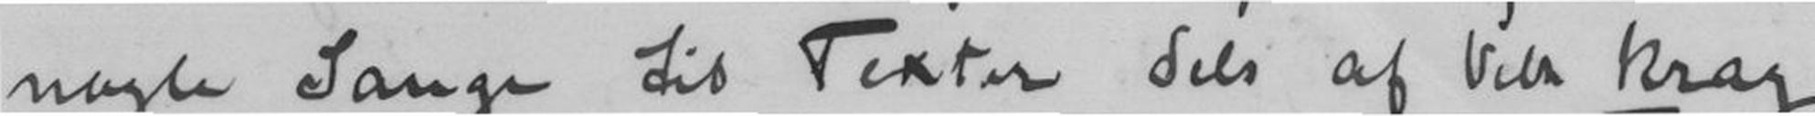nogle Sange til Texter dels af Vilh. Krag
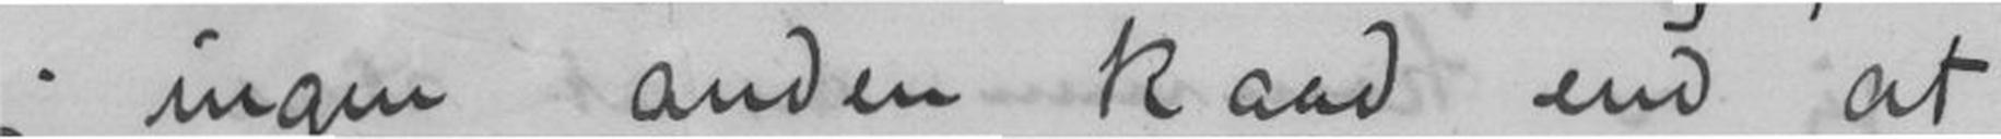jeg ingen anden Raad end at
